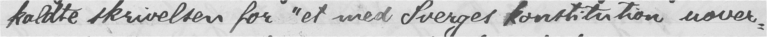kaldte skrivelsen for "et med Sverges konstitution uover-
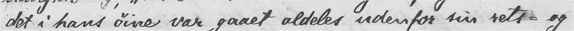det i hans øine var gaaet aldeles udenfor sin rets- og
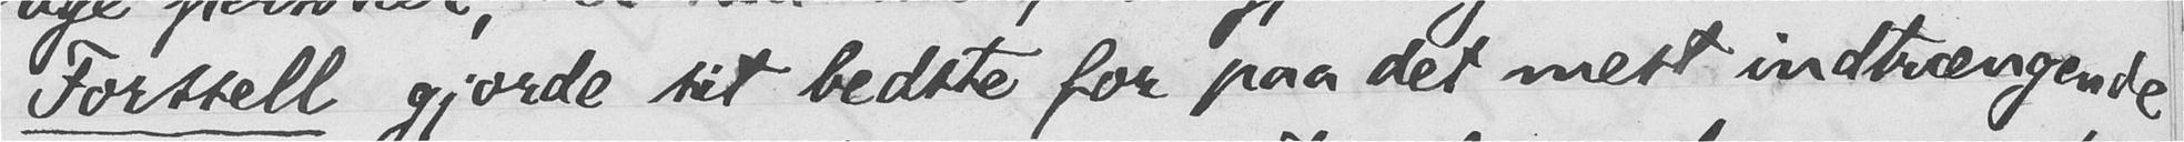Forssell gjorde sit bedste for paa det mest indtrængende
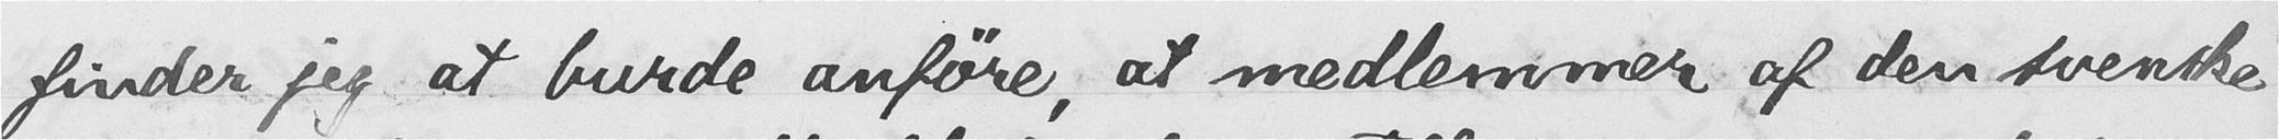finder jeg at burde anføre, at medlemmer af den svenske
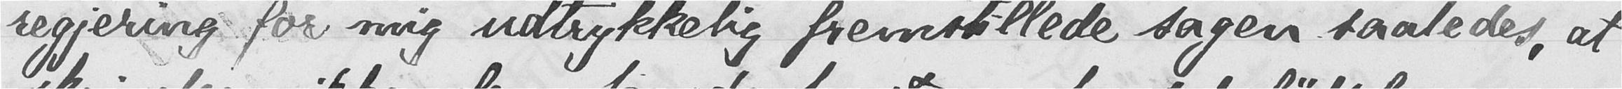regjering for mig udtrykkelig fremstillede sagen saaledes, at
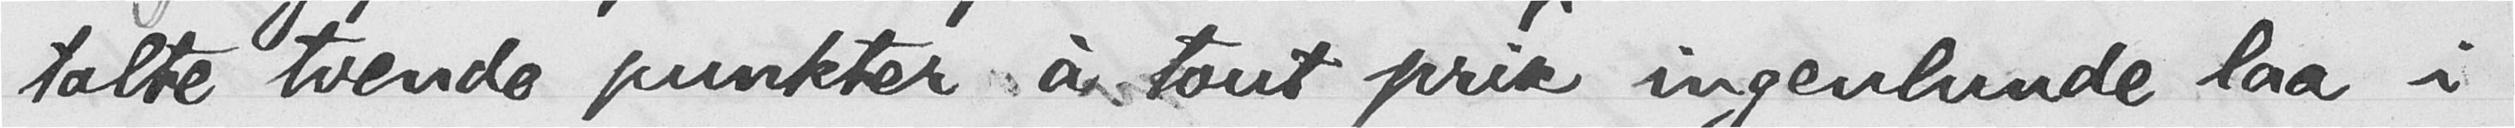talte tvende punkter à tout prix ingenlunde laa i
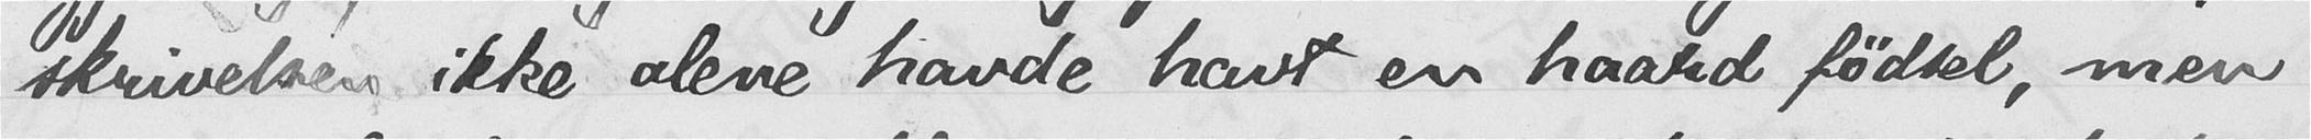skrivelsen ikke alene havde havt en haard fødsel, men
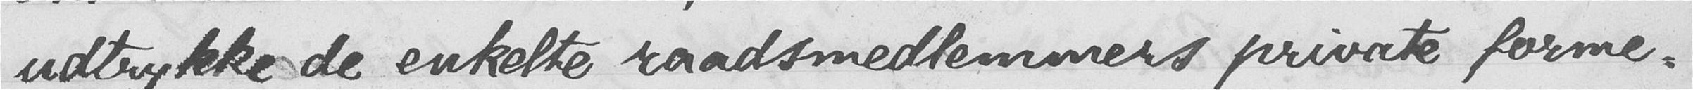udtrykke de enkelte raadsmedlemmers private forme-
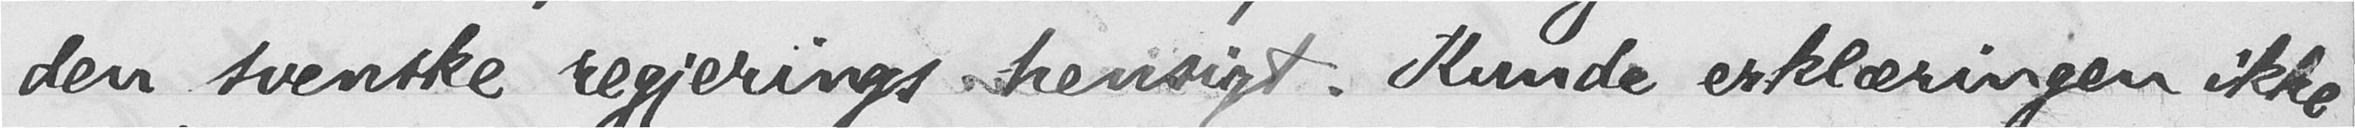den svenske regjerings hensigt. Kunde erklæringen ikke
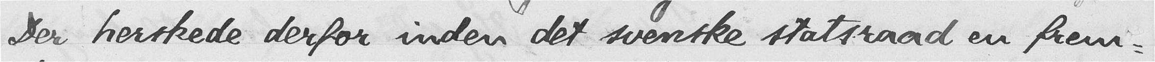Der herskede derfor inden det svenske statsraad en frem-
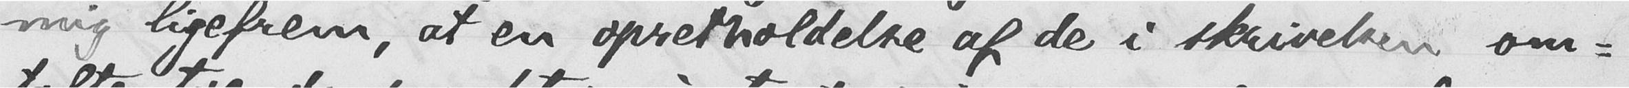mig ligefrem, at en opretholdelse af de i skrivelsen om-
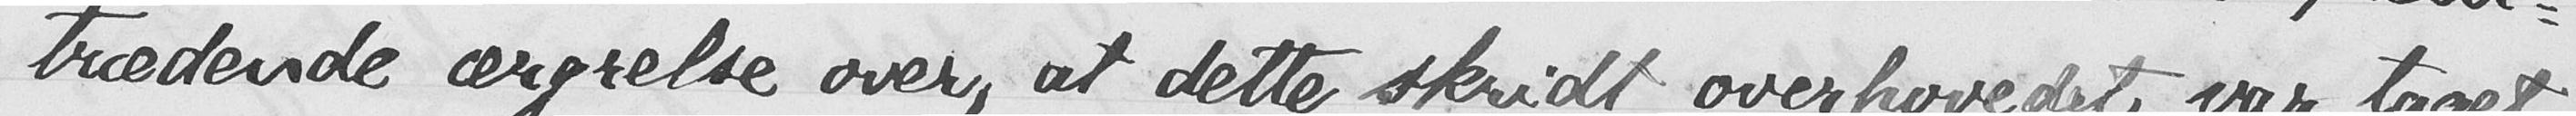trædende ærgrelse over, at dette skridt overhovedet var taget,
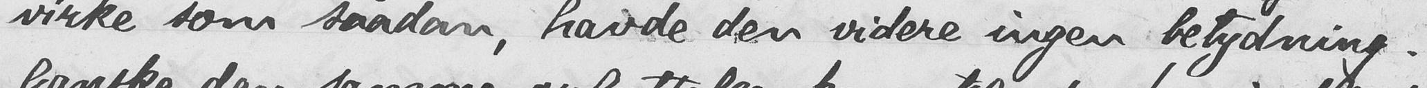virke som saadan, havde den videre ingen betydning.
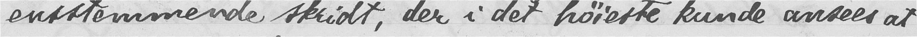ensstemmende skridt, der i det høieste kunde ansees at
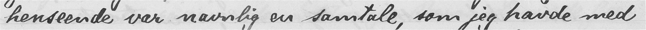henseende var navnlig en samtale, som jeg havde med
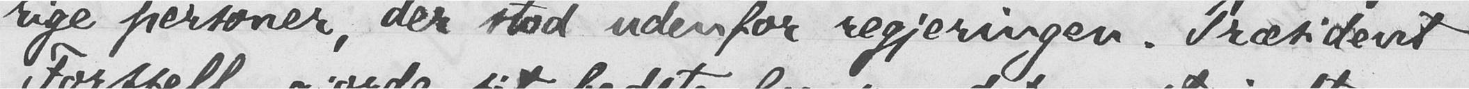rige personer, der stod udenfor regjeringen. Præsident
